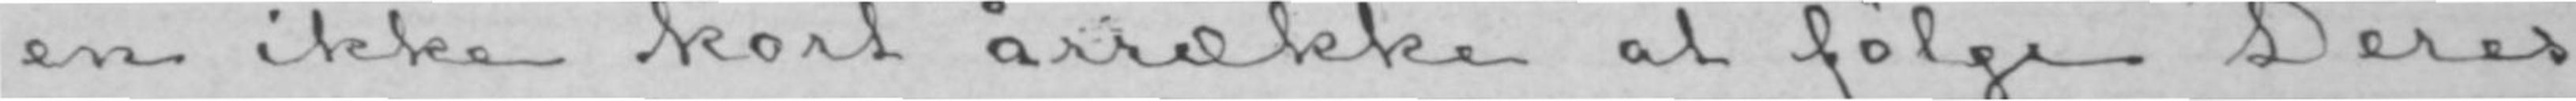en ikke kort årrække at følge Deres
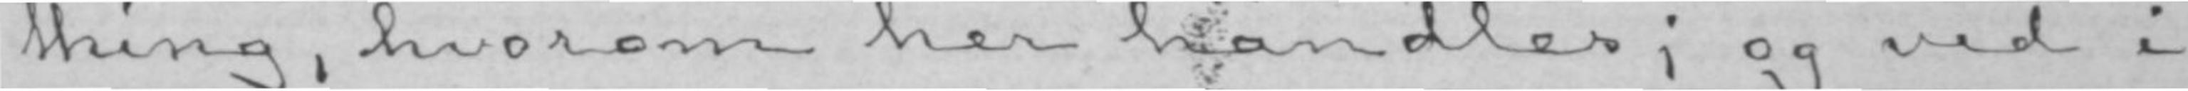thing, hvorom her handles; og ved i
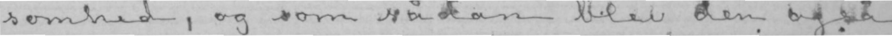somhed, og som sådan blev den også
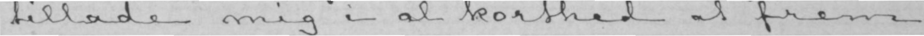tillade mig i al korthed at frem-
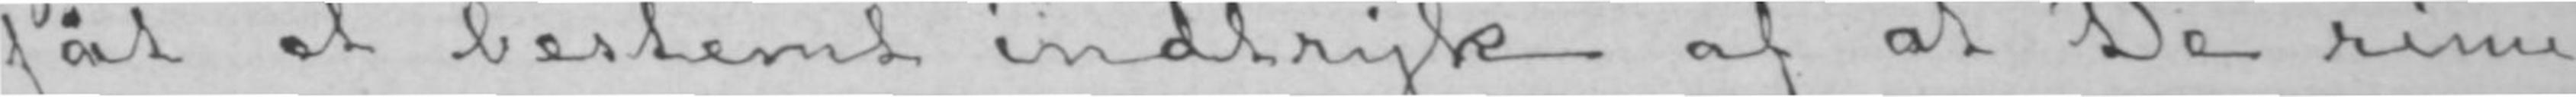fåt et bestemt indtryk af at De rime-
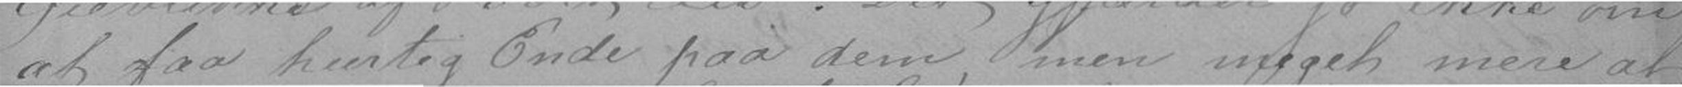at faa hurtig Ende paa dem, men meget mere at
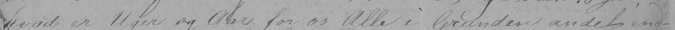hvad er Uger og Aar for os Alle i Grunden andet end
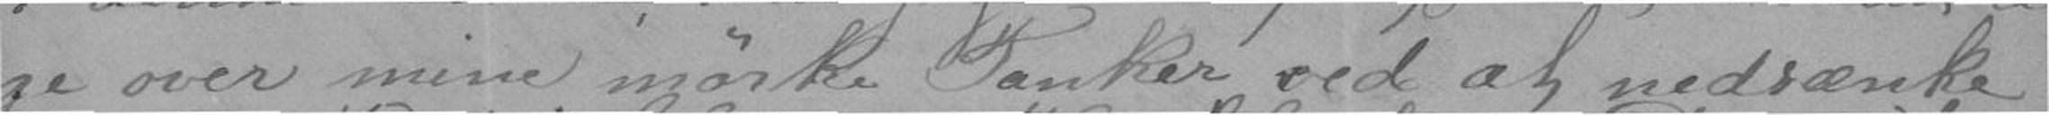ge over mine mørke Tanker ved at nedsænke
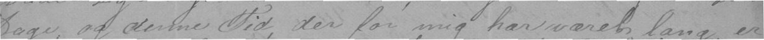Dage, og denne Tid, der for mig har været lang, er
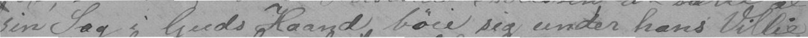sin Sag i Guds Haand bøie sig under hans Villie
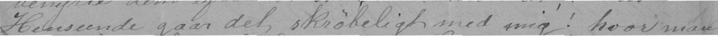Henseende gaar det skrøbeligt med mig! hvor man-
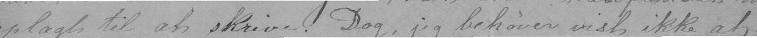oplagt til at skrive. Dog, jeg behøver vist ikke at,
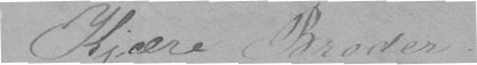Kjære Broder!
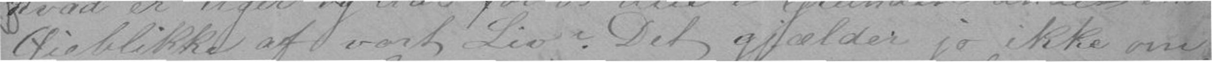Øieblikke af vort Liv? Det gjælder jo ikke om
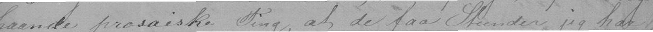haande prosaiske Ting, at de faa Stunder, jeg har
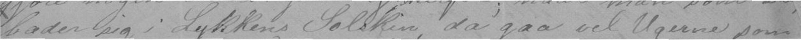bader sig i Lykkens Solskin, da gaa vel Ugerne som
